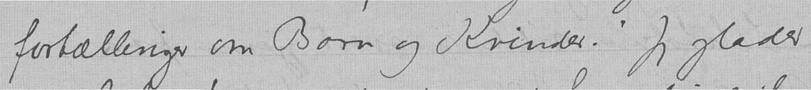fortællinger om Børn og Kvinder." Jeg glæder
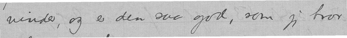vinder, og er den saa god, som jeg tror
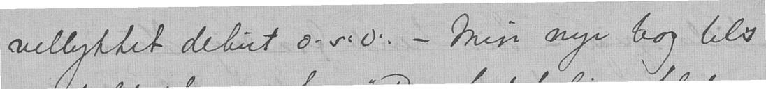vellykket debut o.s.v. — Min nye bog blir
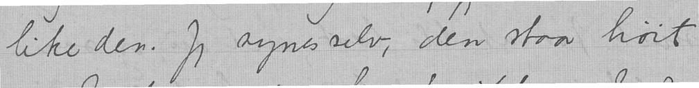like den. Jeg synes selv, den staar høit
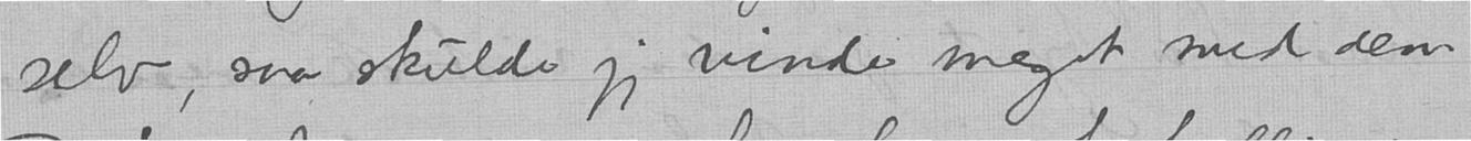selv, saa skulde jeg vinde meget med den.
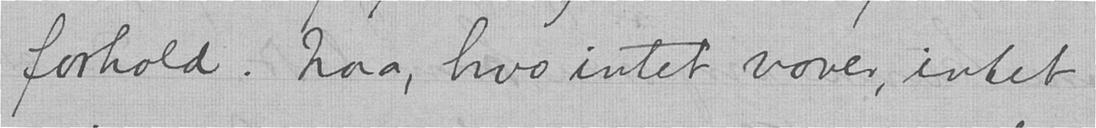forhold. Naa, hvo intet vover, intet
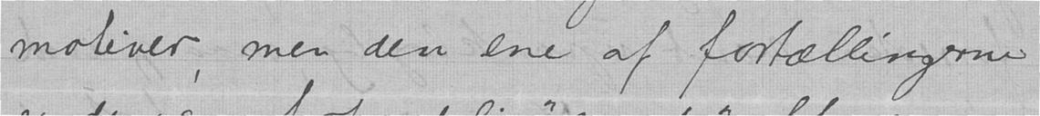motiver, men den ene af fortællingerne
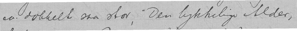ca. dobbelt saa stor, "Den lykkelige Alder,
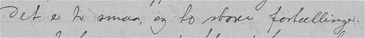Det er to smaa, og to store fortællinger.
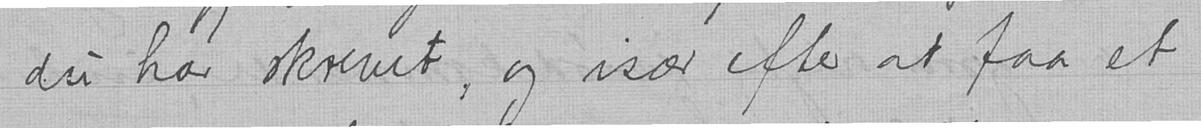du har skrevet, og især efter at faa et
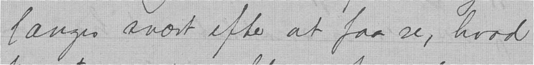Jeg længes svært efter at faa se, hvad
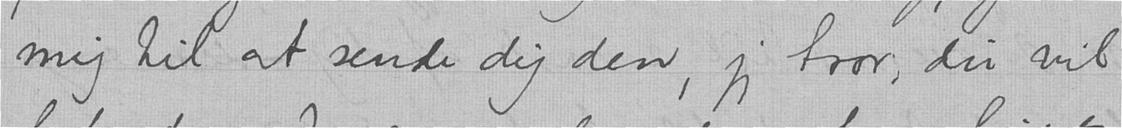mig til at sende dig den, jeg tror, du vil
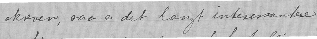skreven, saa er det langt interessantere
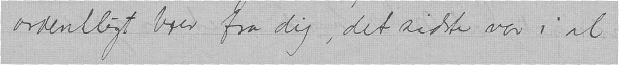ordentligt brev fra dig, det sidste var i al
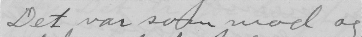Det var som mod og
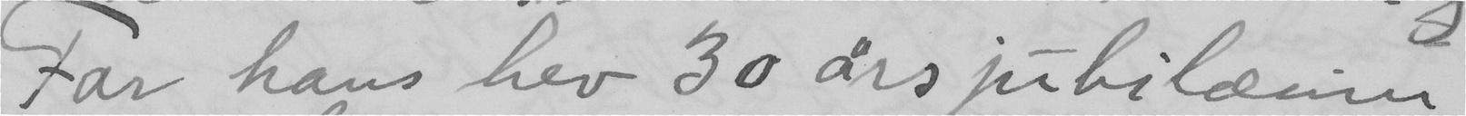Far hans hev 30 årsjubilæum
